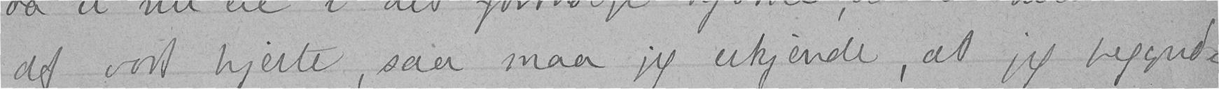af vort hjerte, saa maa jeg erkjende, at jeg begynd-
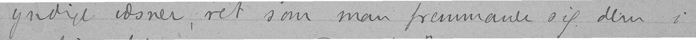yndige væsner, ret som man fremmaner sig dem i
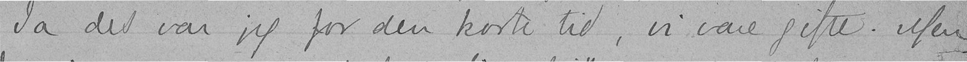Ja det var jeg for den korte tid, vi vare gifte. Men
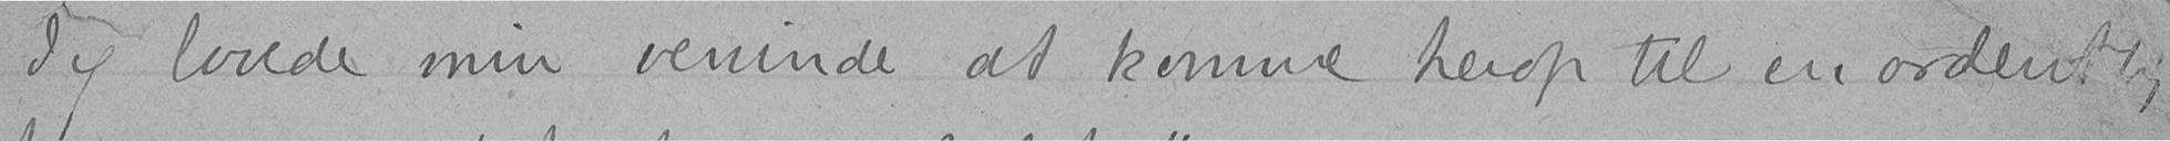Jeg lovede min veninde at komme herop til en ordentlig
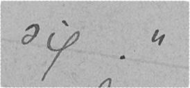sig."
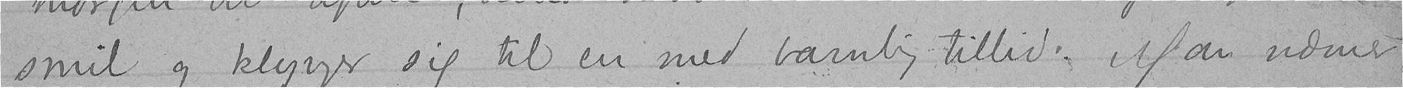smil og klynger sig til en med barnlig tillid. Man nævner
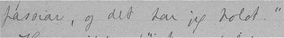passiar, og det har jeg holdt."
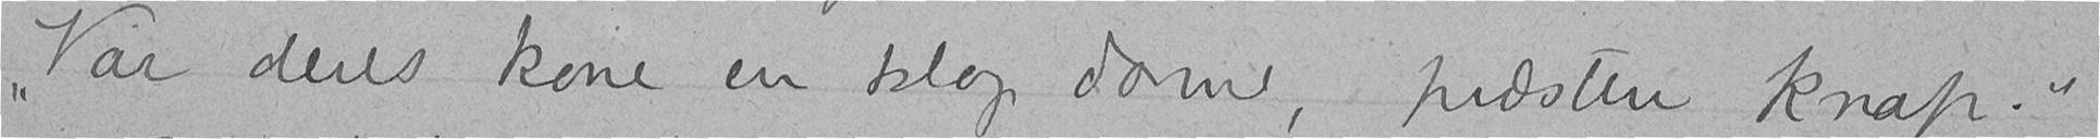"Var deres kone en klog dame, præsten Knop."
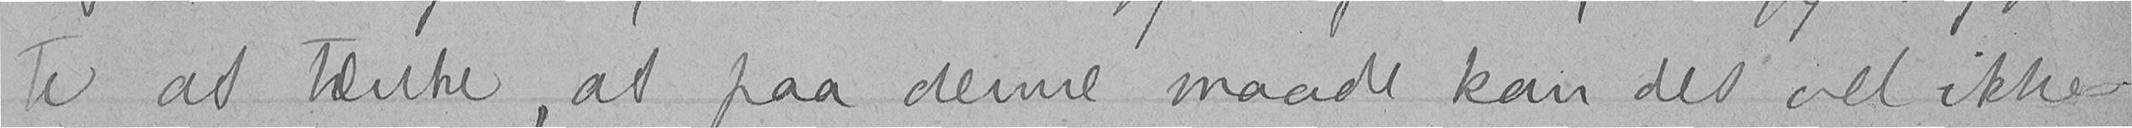te at tænke, at paa denne maade kan det vel ikke
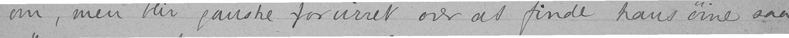om, men blir ganske forvirret over at finde hans øine saa
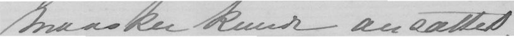Maaske kunde ansættes
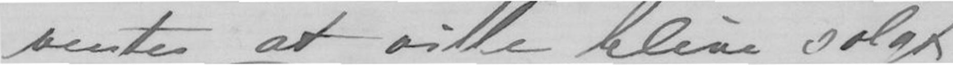ventes at ville blive solgt
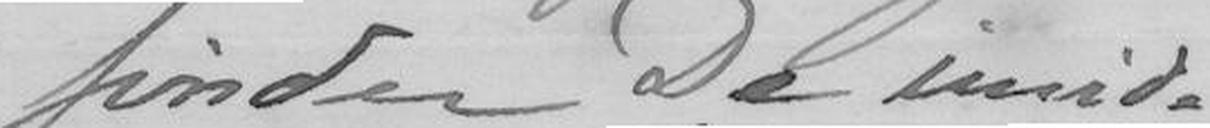finder De imid-
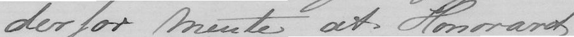derfor Mente at Honoraret
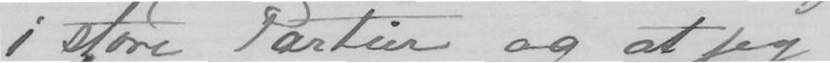i store Partier og at jeg
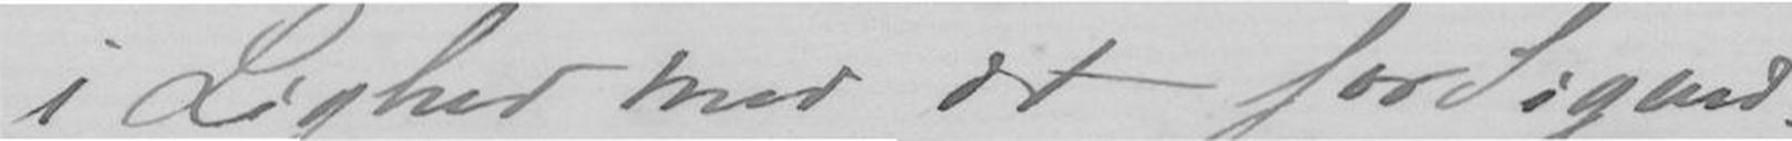i Lighed med det for Sigurd
